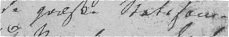de græske Statssam-
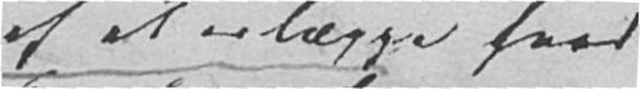af at erlægge hvad
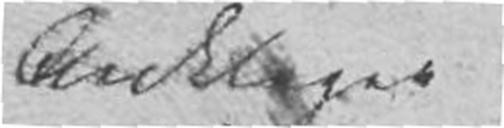Anklager
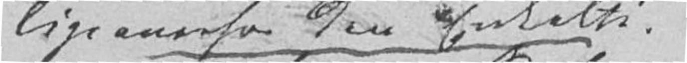ligeoverfor den Enkelte.
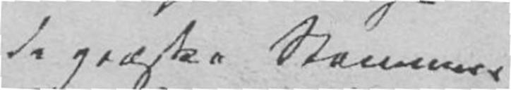de græske Stammers
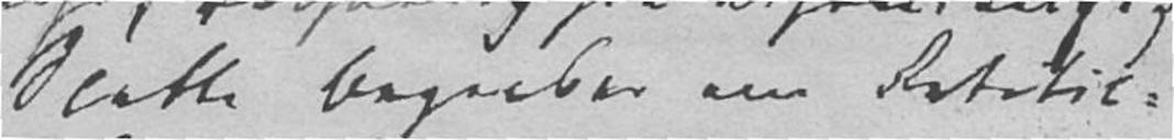Slette begreber om Retstil-
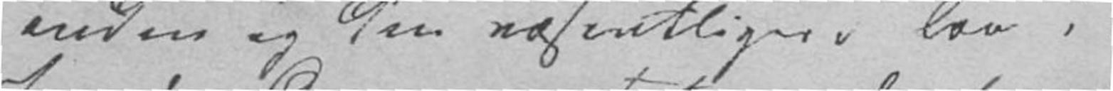anden og den væsentligere laa i
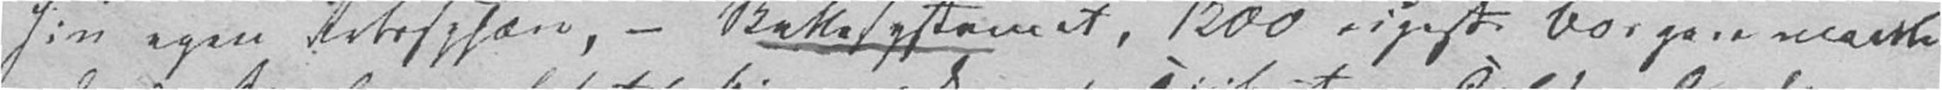sin egen Retssphære, - Skattesystemet, 1200 rigeste Borgere maatte
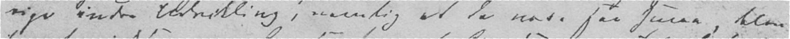rige indre Udvikling, nemlig at de vare saa smaa, blev
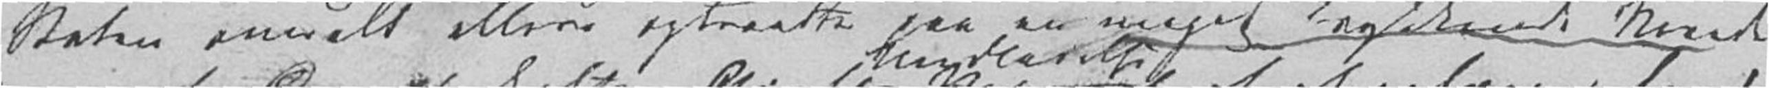Staten overalt ellers optraadte paa en meget trykkende Maade
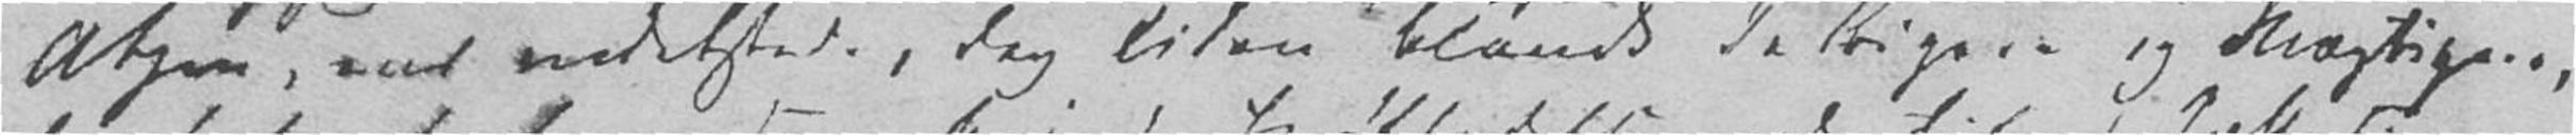Athen, end andetsteds, dog liden blandt de Rigere og Mægtigere,
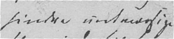hindre uretmæssige
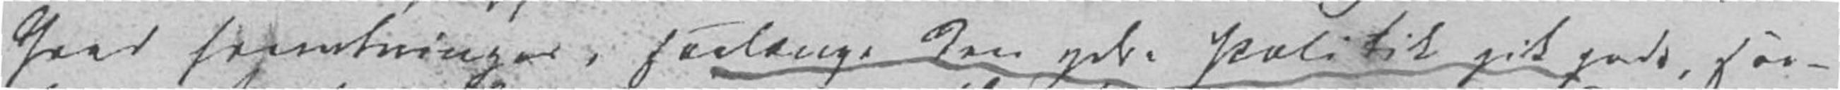Grad fremtvinges, saalænge den ydre Politik gik godt, saa-
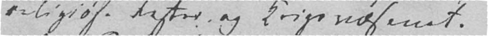religiøse Fester og Krigsvæsenet.
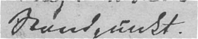Standpunkt.
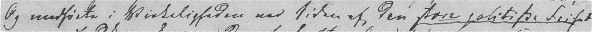Og medførte i Virkeligheden ved Siden af den store politiske Frihed
